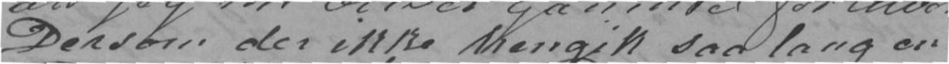Dersom der ikke hengik saa lang en
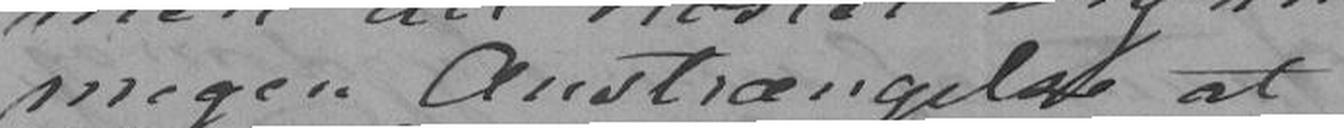megen Anstrængelse at
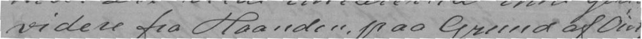videre fra Haanden paa Grund af Øiet,
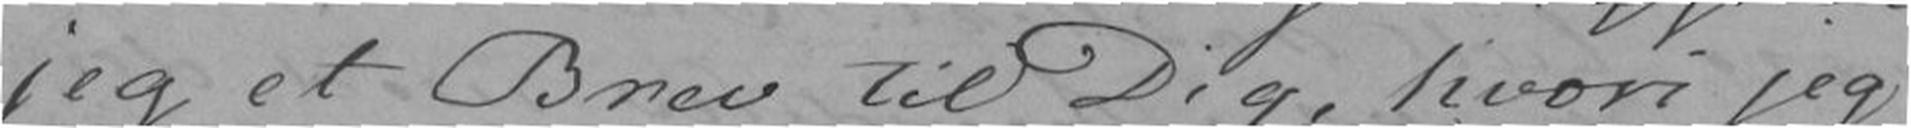jeg et Brev til Dig, hvori jeg
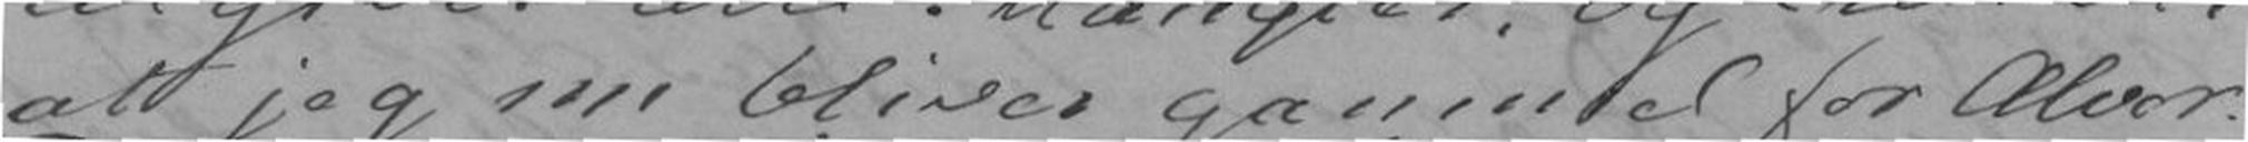at jeg nu bliver gammel for Alvor.
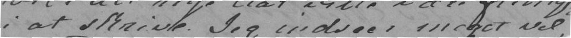i at skrive. Jeg indseer meget vel
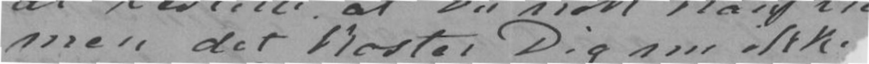men det koster Dig nu ikk
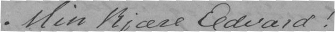Min kjære Edvard!
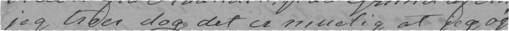jeg troer dog det er muelig at jeg og-
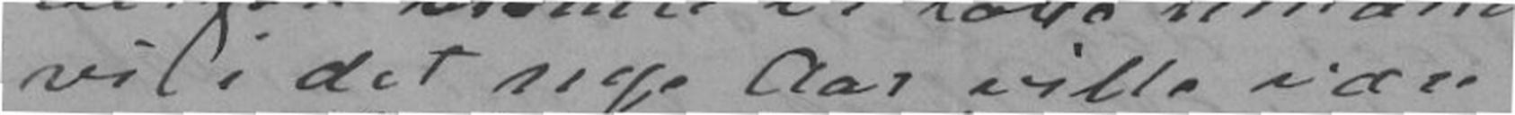vi i det nye Aar ville være
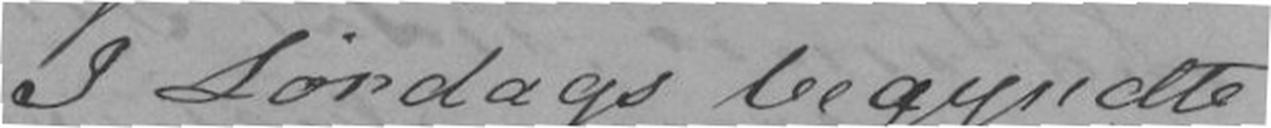I Lørdags begyndte
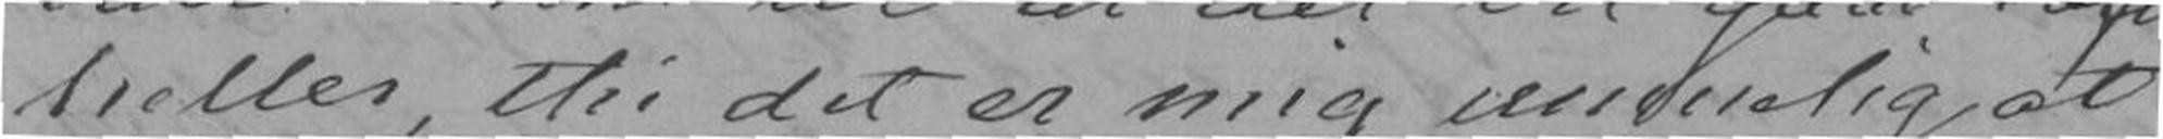heller, thi det er mig umuelig at
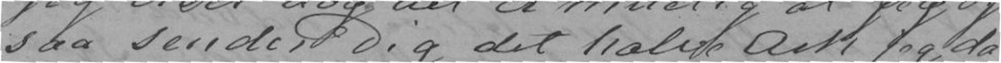saa sender Dig det halve Ark jeg da
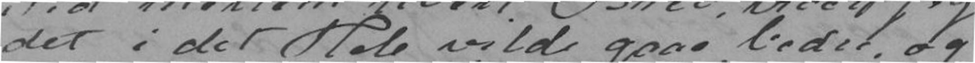det i det Hele vilde gaae bedre, og
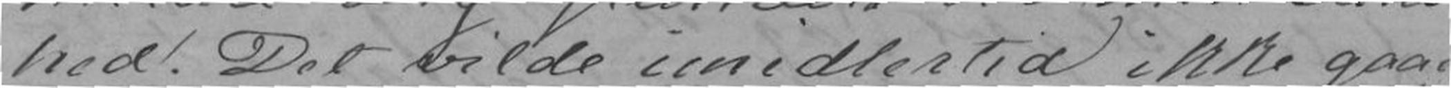hed! Det vilde imidlertid ikke gaae
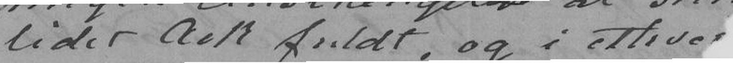lidet Ark fuldt, og i ethver
